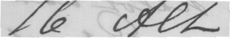16 Alt
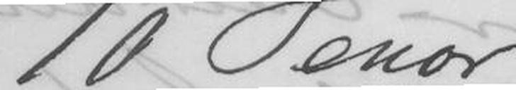10 Tenor Stemmer
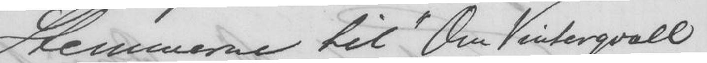Stemmerne til "Om Vinterqvæll
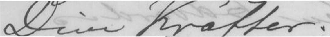Dine Kræfter.
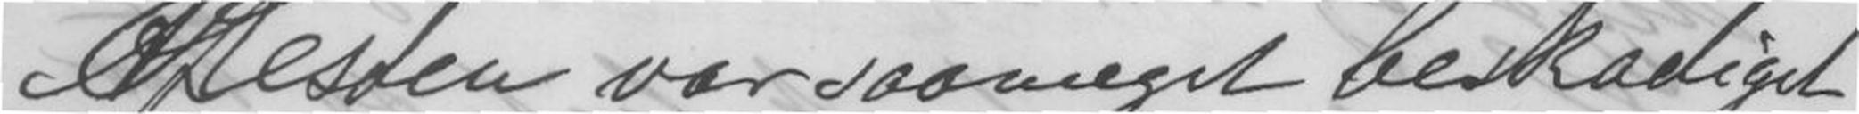Resten var saameget beskadiget
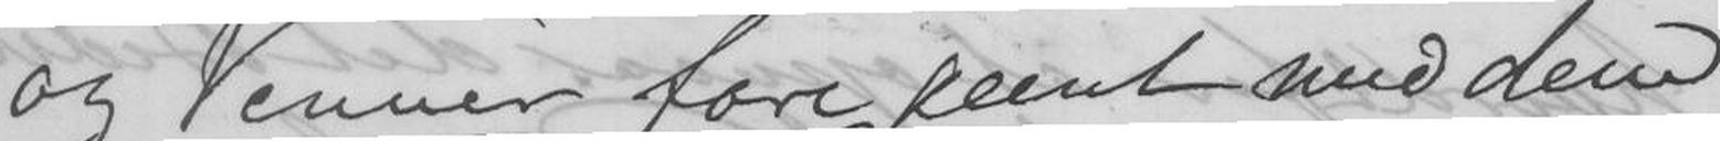og Venner fare pent med dem
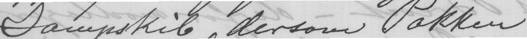Dampskib, dersom Pakken
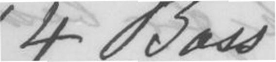14 Bass
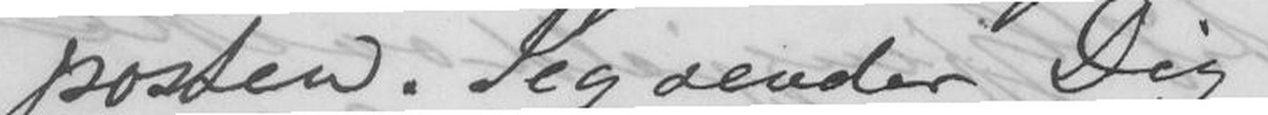posten. Jeg sender Dig
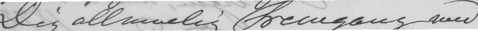Dig allmenlig Fremgang med
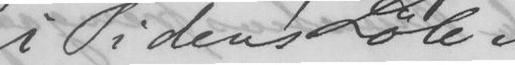i Tidens Løb.
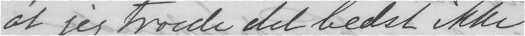at jeg troede det bedst ikke
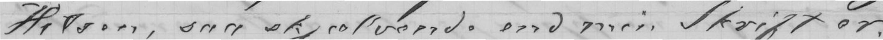Hilsen, saa skjælvende end min Skrift er.
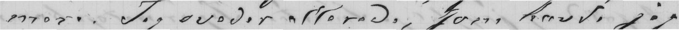mere. Jeg sveder allerede, som havde jeg
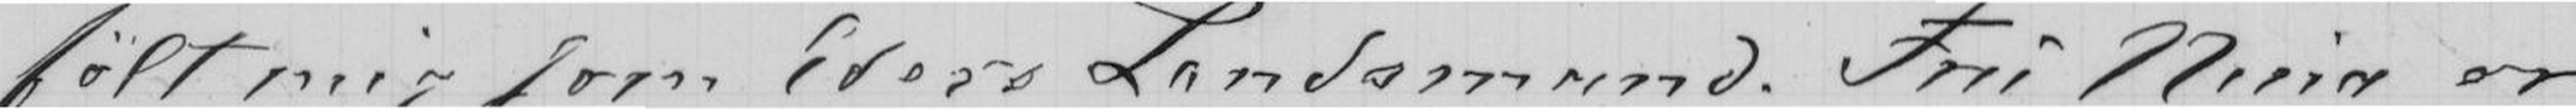følt mig som Eders Landsmand. Fru Nina er
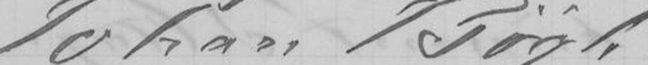Johan Bøgh
